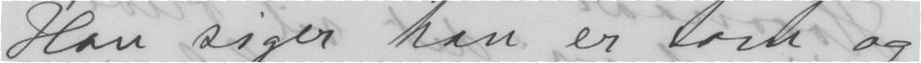Han siger han er tom og
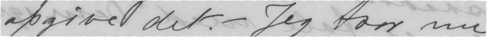opgive det.- Jeg tror nu
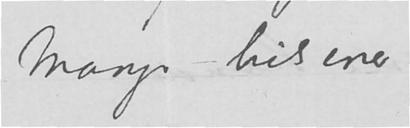Mange hilsener
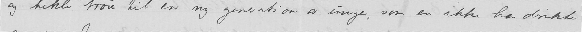og hekle trøier til en ny generation av unger, som en ikke har direkte
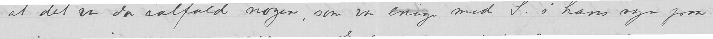at det var da ialfald nogen, som var enig med S. i hans syn paa
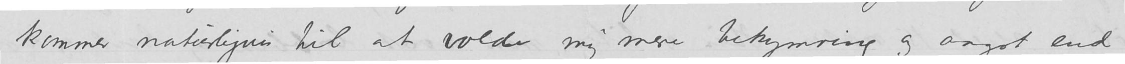kommer naturligvis til at volde mig mere bekymring og angst end
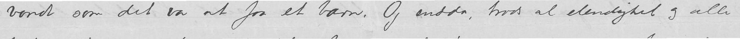vondt som det var at faa et barn. Og endda, trods al elendigtet og alle
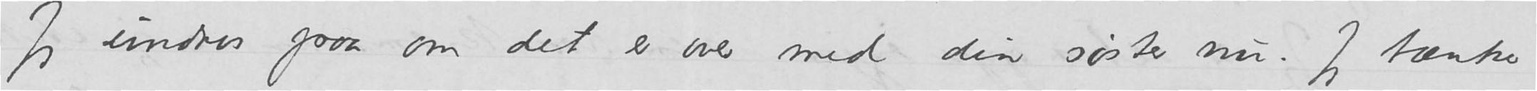Jeg undres paa om det er over med din søster nu. Jeg tænker
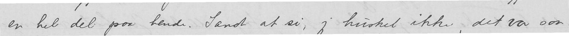en hel del paa hende. Sandt at si, jeg husket ikke, det var saa
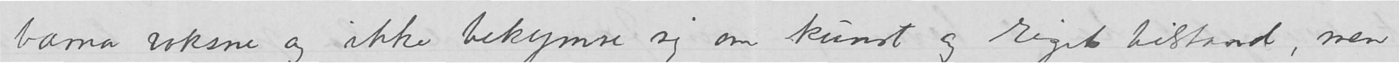barna voksne og ikke bekymre sig om kunst og rigets tilstand, men
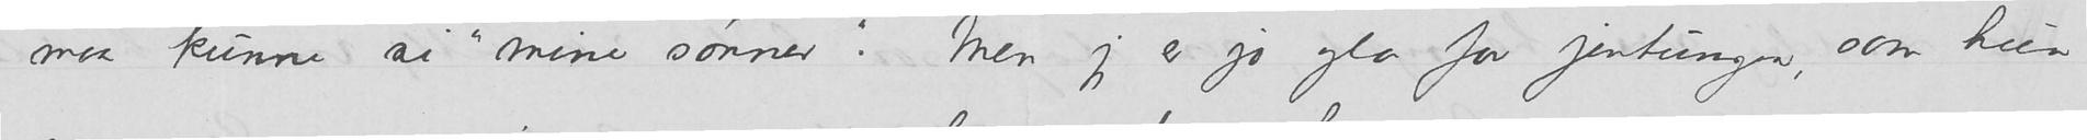maa kunne si «mine sønner». Men jeg er jo gla for jentungen, som hun
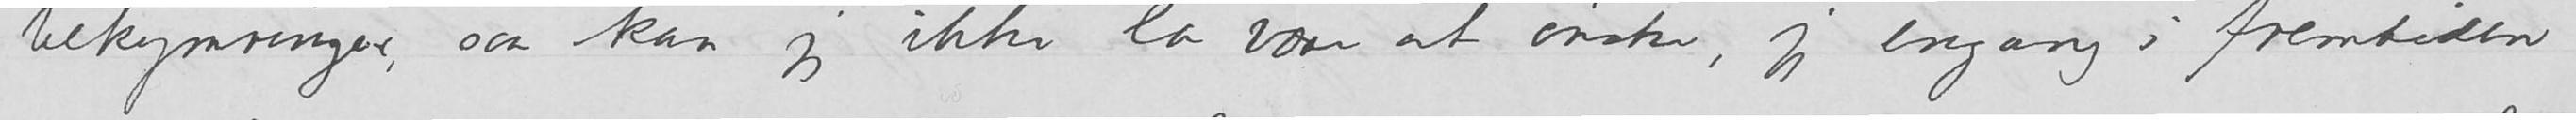bekymringer, saa kan jeg ikke la være at ønske, jeg engang i fremtiden
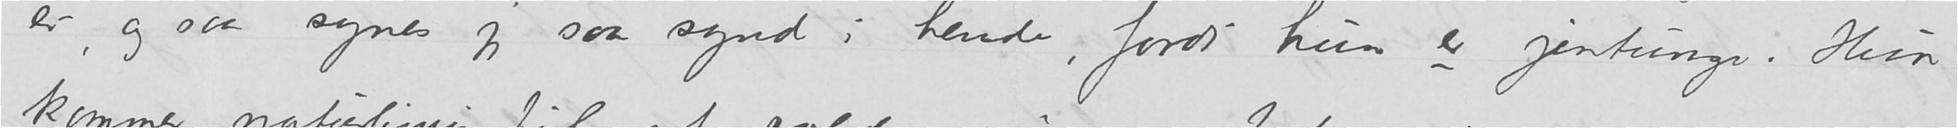er, og saa synes jeg saa synd i hende, fordi hun er jentunge. Hun
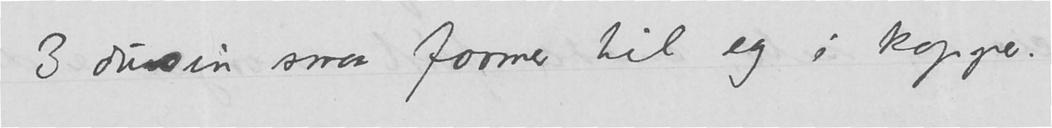3 dusin smaa former til og i kopper.
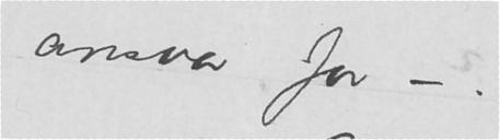ansvar for –.
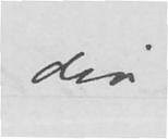din
